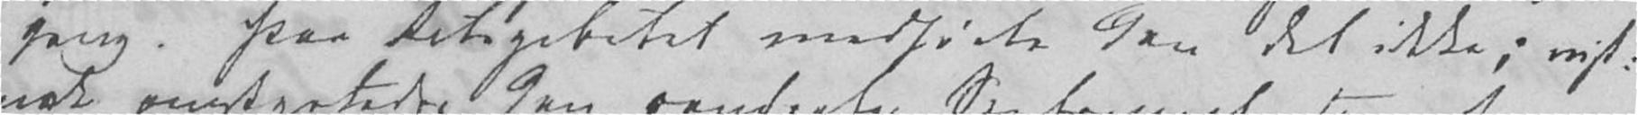gang. Paa Retsgebetet medførte den det ikke; vist-
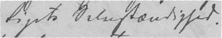Rigets Selvstændighed.
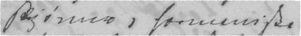skjønne, harmoniske
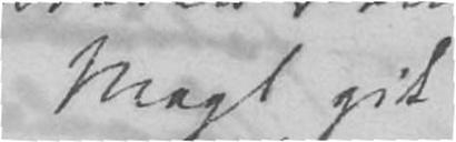Magt gik
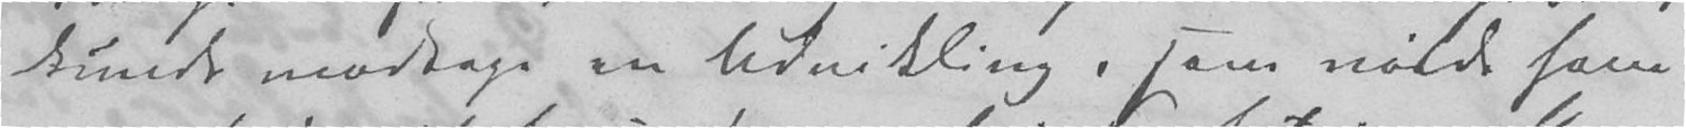kunde modtage en Udvikling, som vilde have
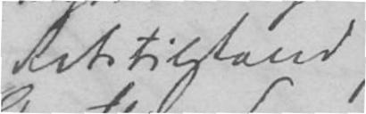Retstilstand
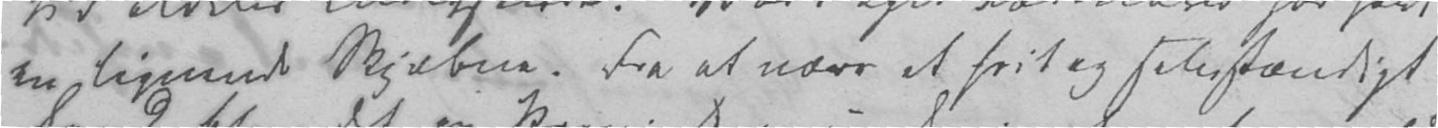en lignende Skjæbne. Fra at være et frit og selvstændigt
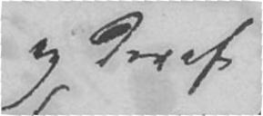og deraf
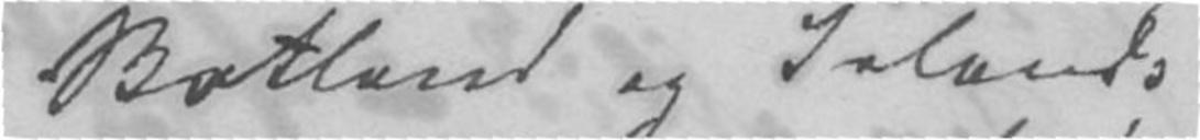Skottland og Islands
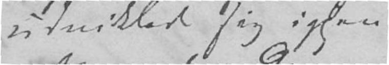udviklede sig igjen
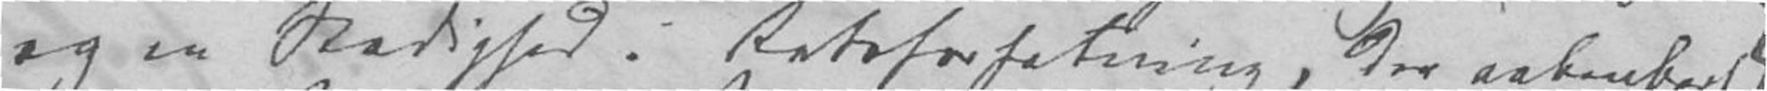og en Stadighed i Retsforfatning, der aabenbart
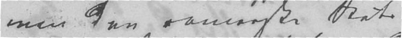men den romerske Stats
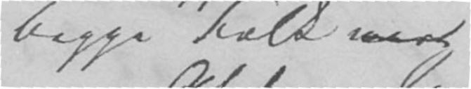begge Folk
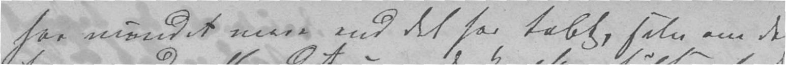har vundet mere end det har tabt, selv om de
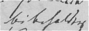bibeholdtes
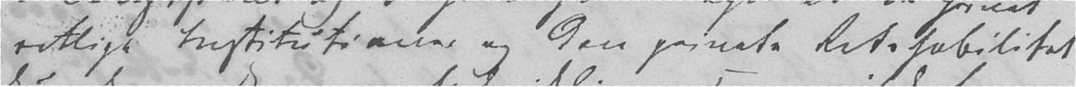retlige Institutioner og den Private Retshabilitet
